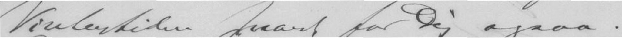Vintertiden snart for Dig ogsaa.
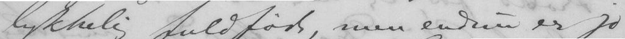lykkelig fuldført, men endnu er jo
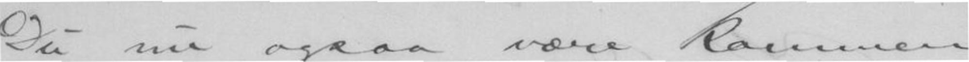Du nu ogsaa være kommen
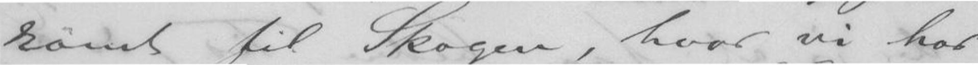rømt til Skogen, hvor vi har
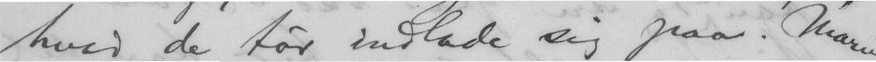hvad de tør indlade sig paa. Marie,
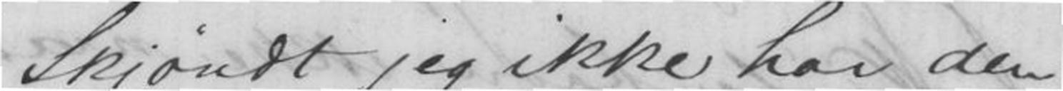Skjøndt jeg ikke har den
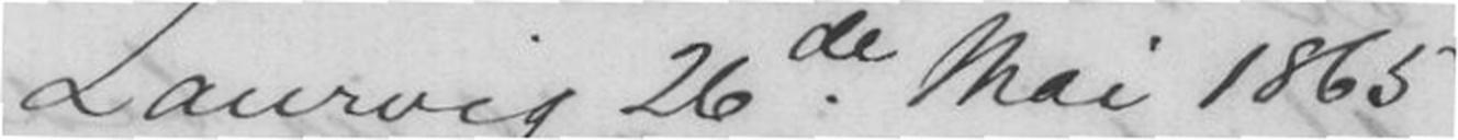Laurvig 26de Mai 1865
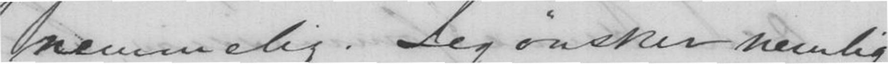nemmelig. Jeg ønsker nemlig
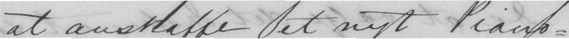at anskaffe et nyt Piano-
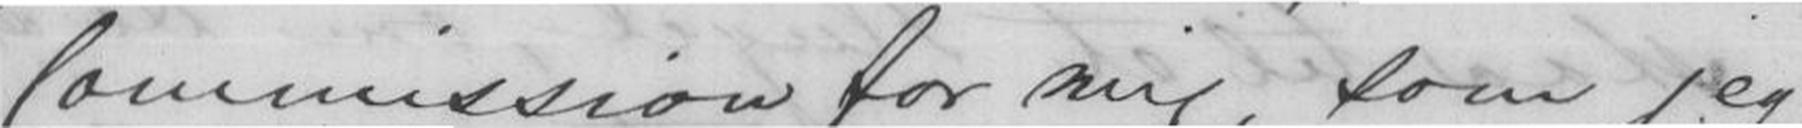Commission for mig, som jeg
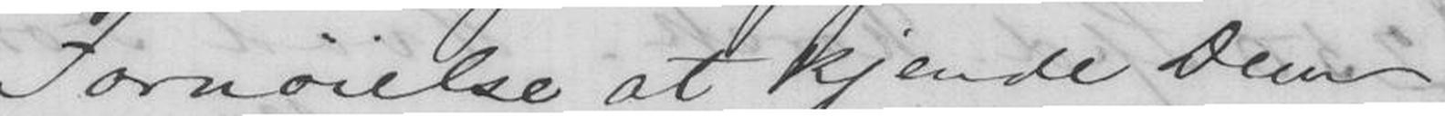Fornøielse at kjende Dem
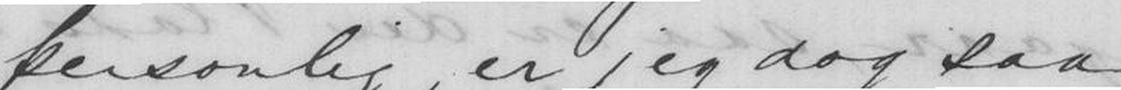Pesonlig, er jeg dog saa
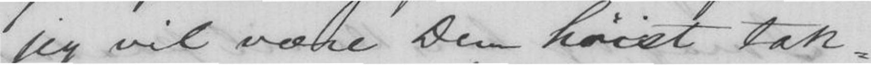jeg vil være Dem høist tak-
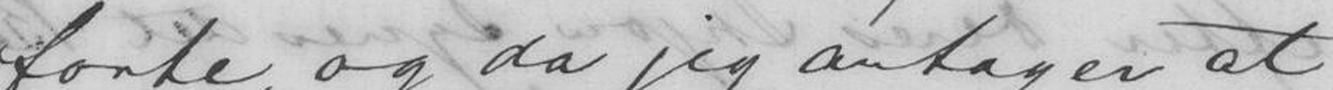forte, og da jeg antager at
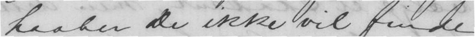haaber De ikke vil finde
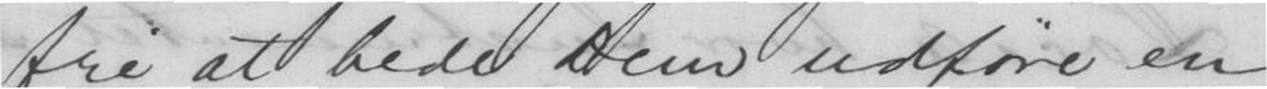fri at bede Dem udføre en
